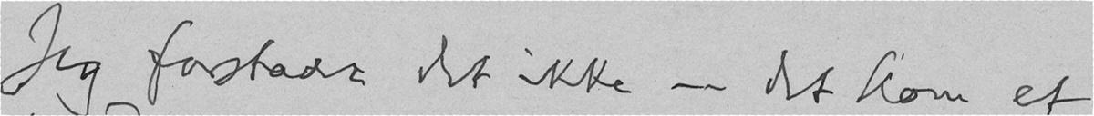Jeg forstaar det ikke - det kom et
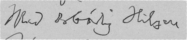Med ærbødig Hilsen
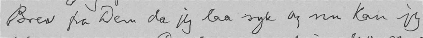Brev fra Dem da jeg laa syk og nu kan jeg
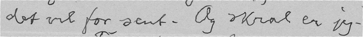det vel for sent. Og skral er jeg.
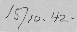15/10. 42.
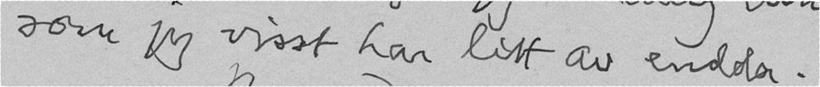som jeg visst har litt av endda.
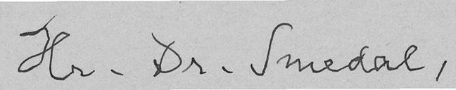Hr. Dr. Smedal,
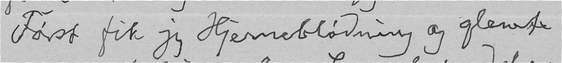Først fik jeg Hjerneblødning og glemte
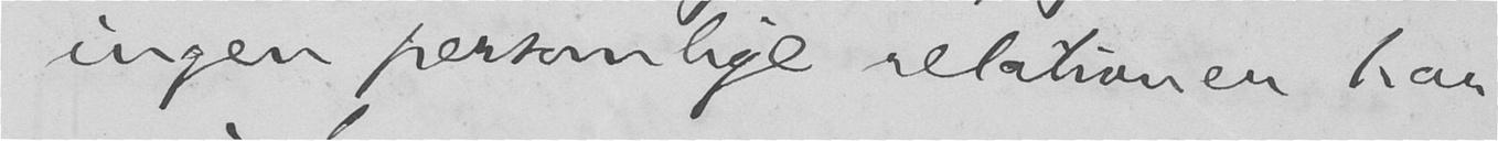ingen personlige relationer har
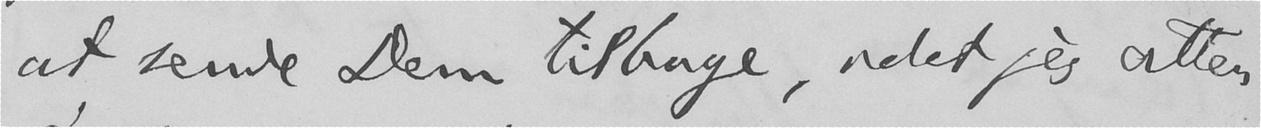at sende Dem tilbage, idet jeg atter
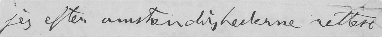jeg efter omstændighederne rettest
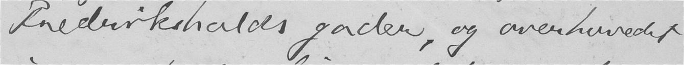Fredrikshalds gader, og overhovedet
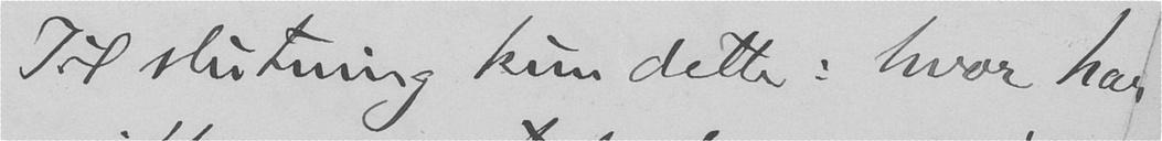Til slutning kun dette: hvor har
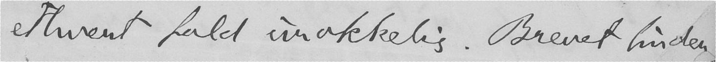ethvert fald urokkelig. Brevet finder
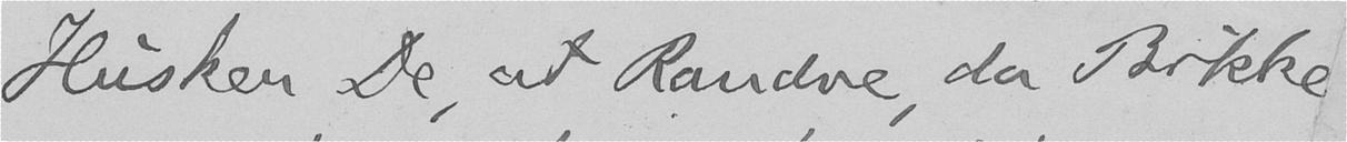Husker De at Randve, da Bikke
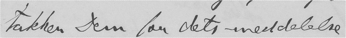takker Dem for dets meddelelse.
